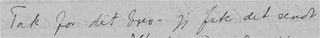Tak for dit brev — jeg fik det sendt
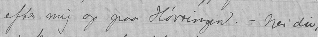efter mig op paa Høvringen. — Nei du,
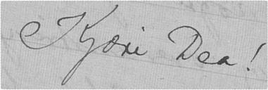Kjære Dea!
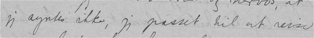jeg syntes ikke, jeg passet til at reise
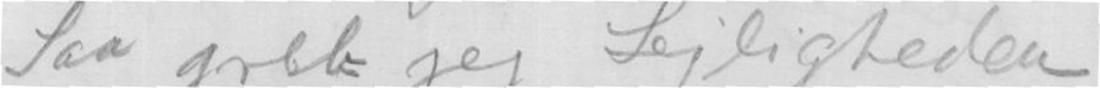Saa greb jeg Lejligheden
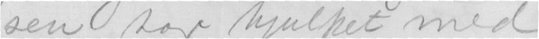sen har hjulpet med
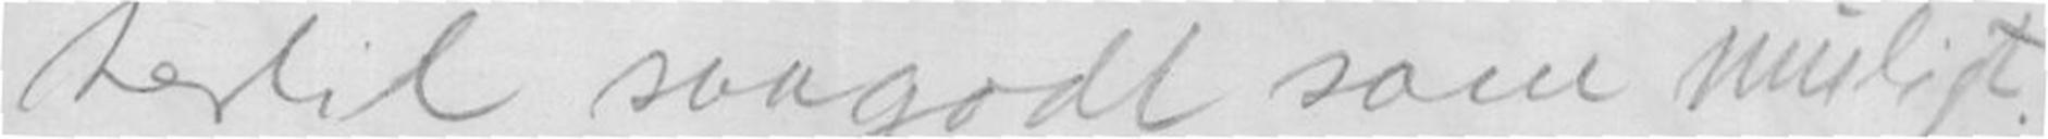hertil saagodt som muligt.
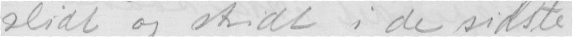slidt og stridt i de sidste
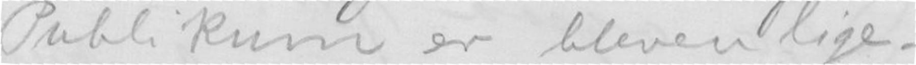Publikum er bleven lige-
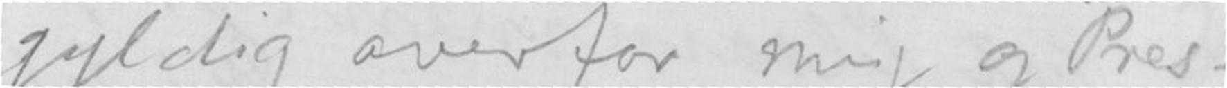gyldig ovenfor mig og Pres-
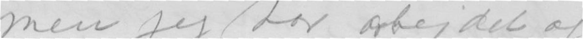men jeg har arbejdet og
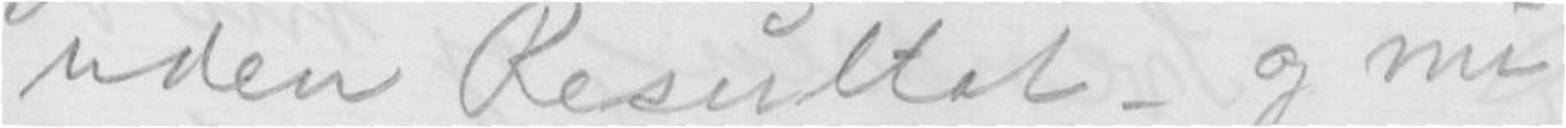uden Resultat - og nu
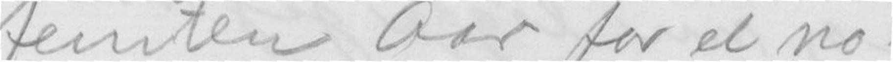femten Aar for et no
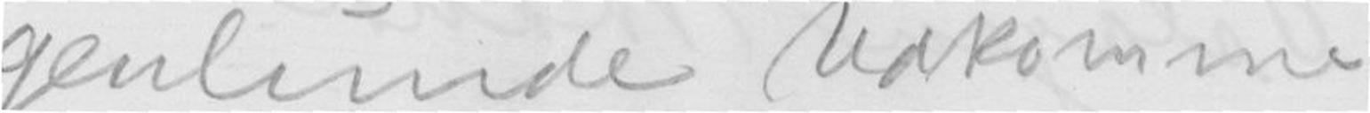genlunde udkomme
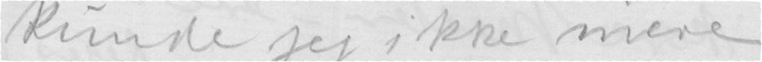kunde jeg ikke mere.
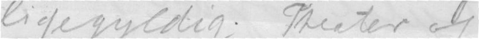ligegyldig. Theater og
